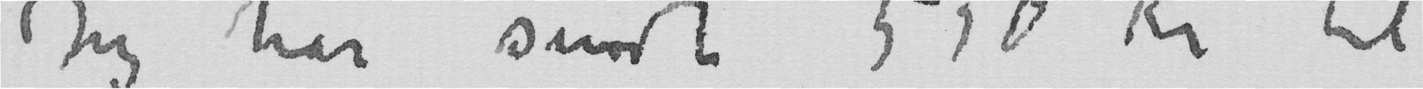Jeg har sendt 550 Kr til
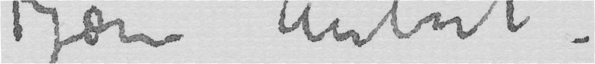Kjære Aubert.
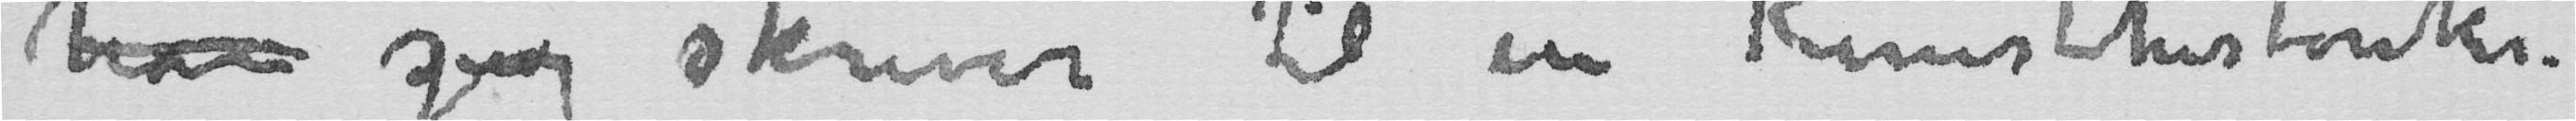han jeg skriver til en Kunsthistoriker.
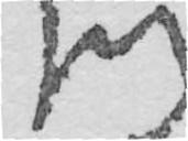jeg
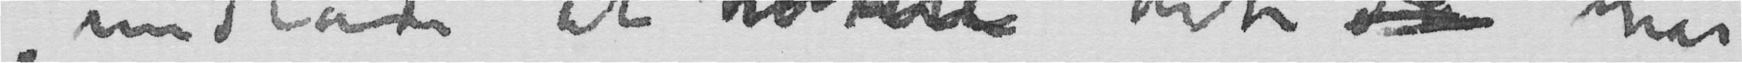undlade at notere det da når
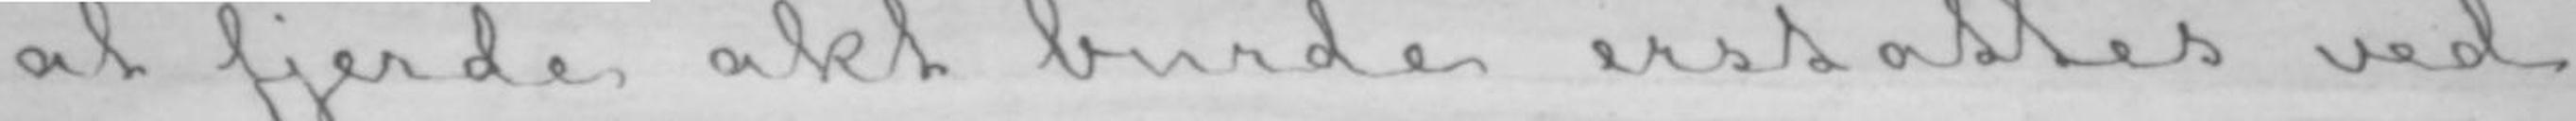at fjerde akt burde erstattes ved
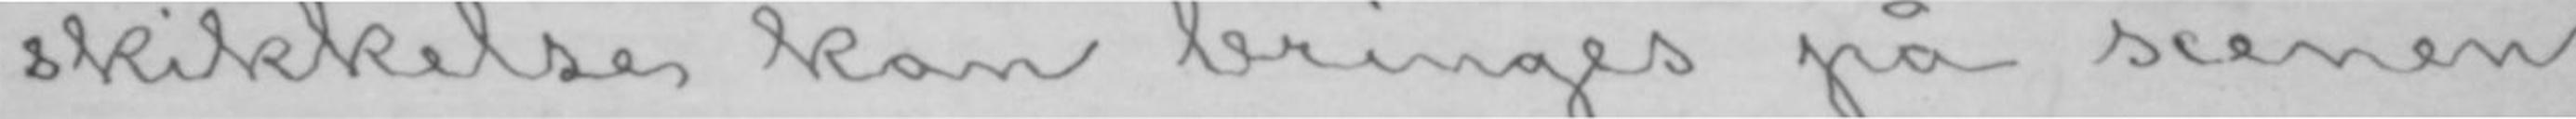skikkelse kan bringes på scenen,
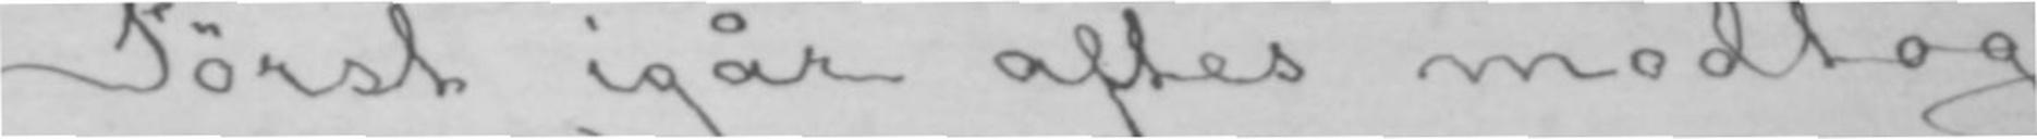Først igår aftes modtog
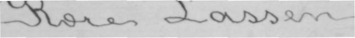Kære Lassen!
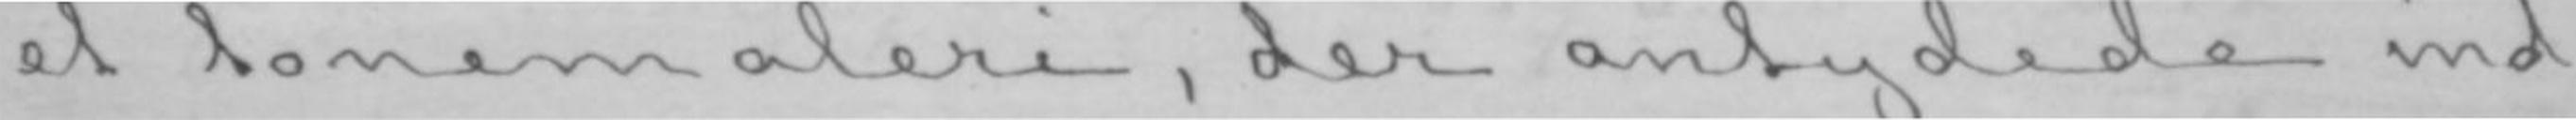et tonemaleri, der antydede ind-
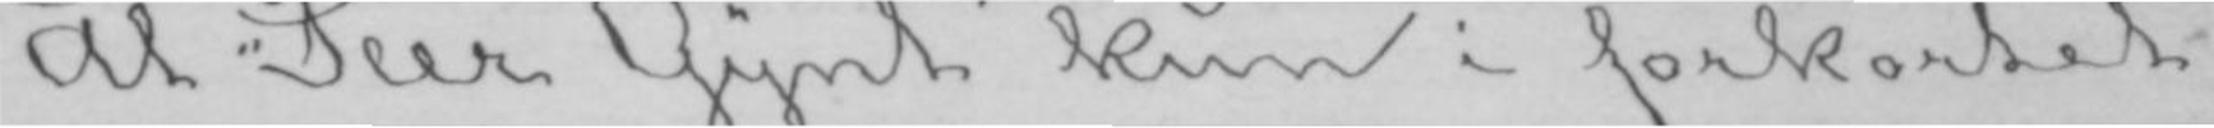At «Peer Gynt» kun i forkortet
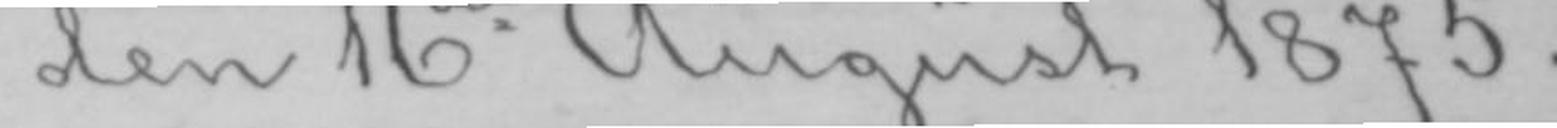den 16de August 1875.
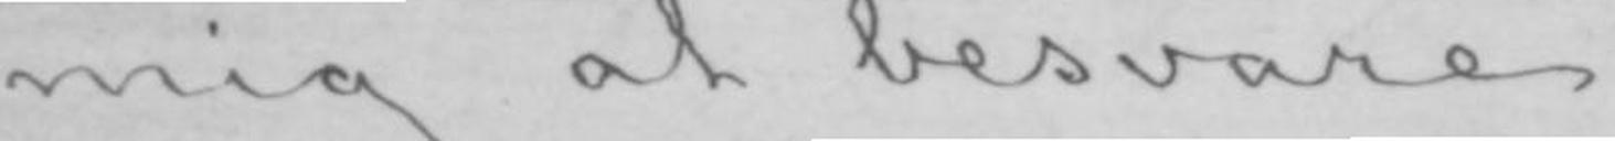mig at besvare.
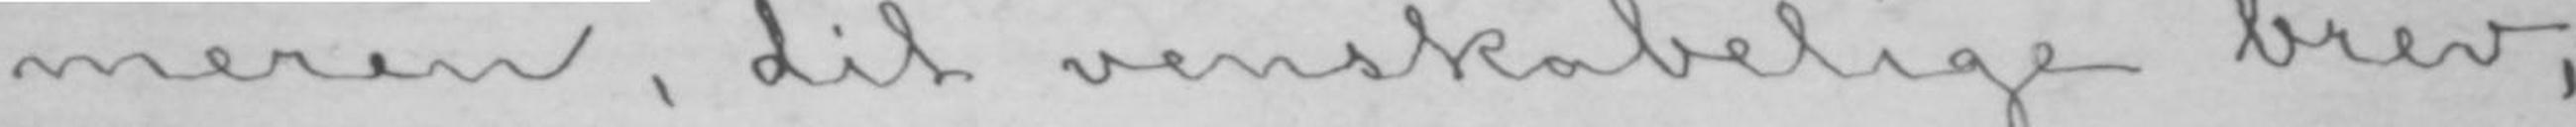meren, dit venskabelige brev,
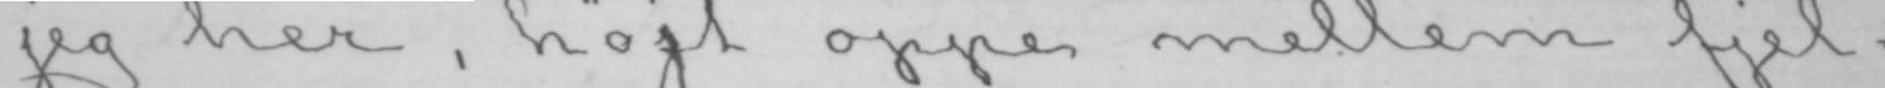jeg her, højt oppe mellem fjel-
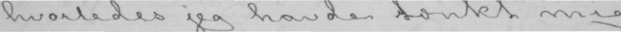hvorledes jeg havde tænkt mig
